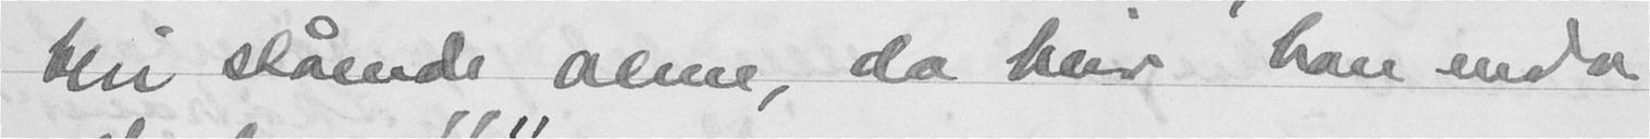blir stående alene, da blir han enda
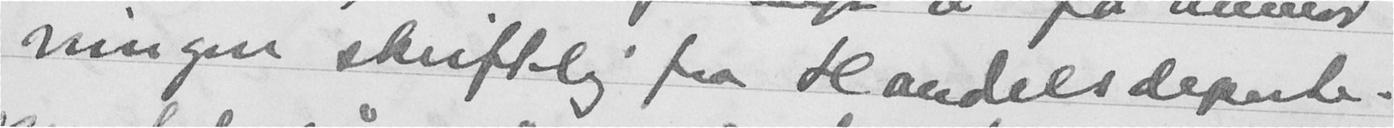ningen skriftlig fra Handelsdeparte-
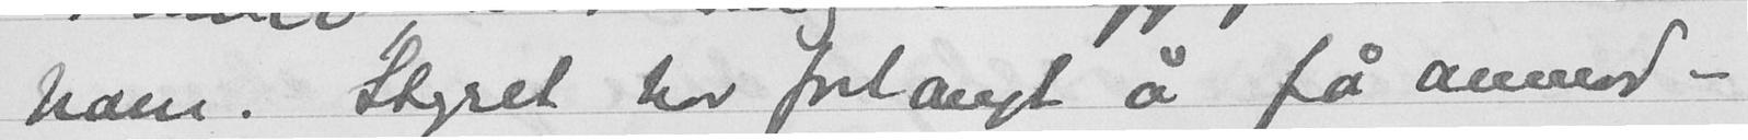ham. Styret har forlangt å få anmod-
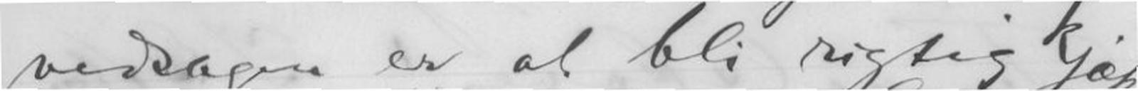vedsagen er at bli rigtig kjæk
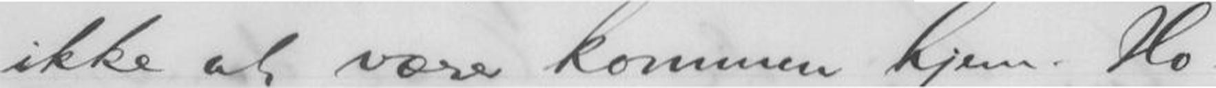ikke at være kommen hjem. Ho-
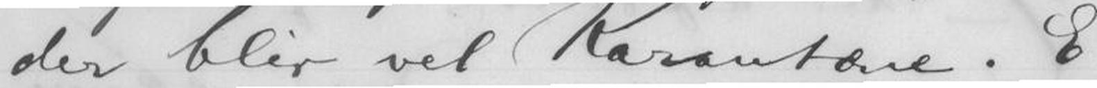der blir vel Karantæne. E-
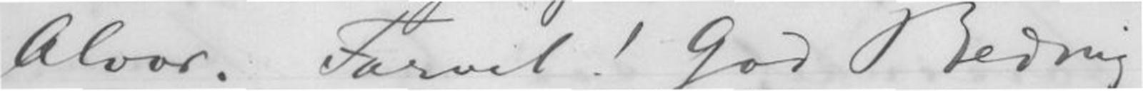Alvor. Farvel! God Bedring
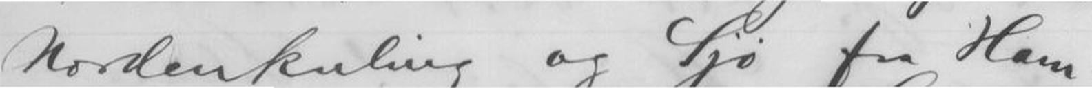Nordenkuling og Sjø fra Ham-
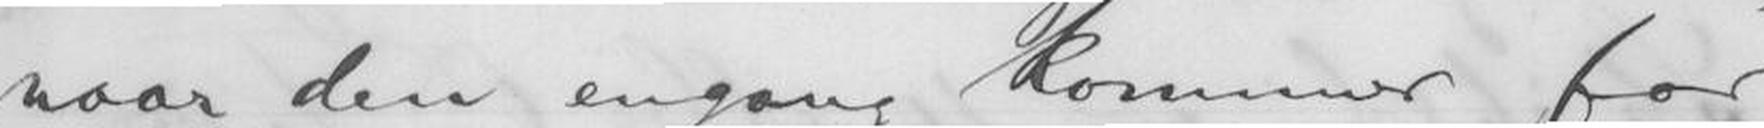naar den engang kommer for
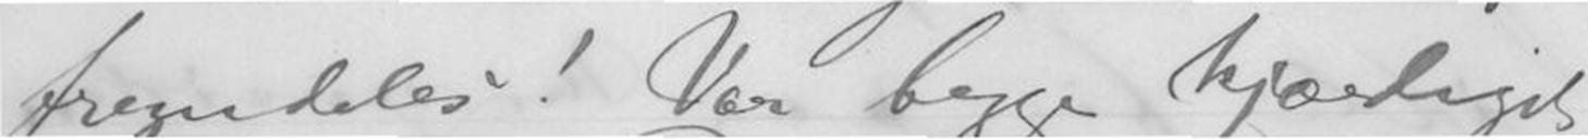fremdeles! Vær begge hjærteligst
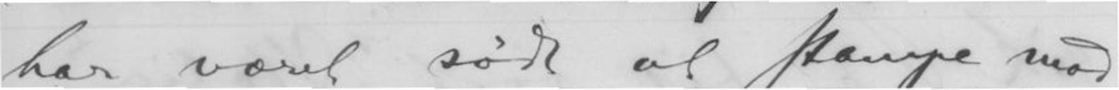har været sødt at stampe mod
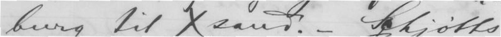burg til Xsand. - Schjøtts
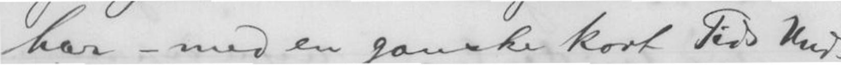har - med en ganske kort Tids Und-
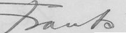Frants.
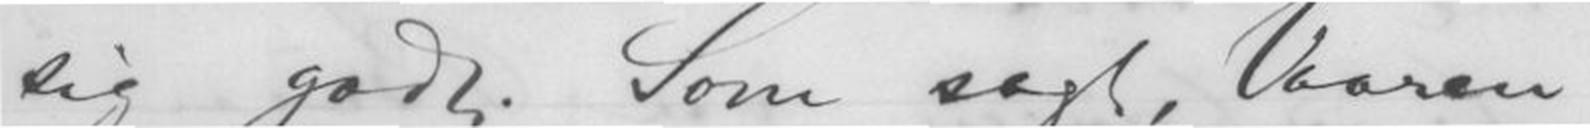sig godt. Som sagt, Vaaren
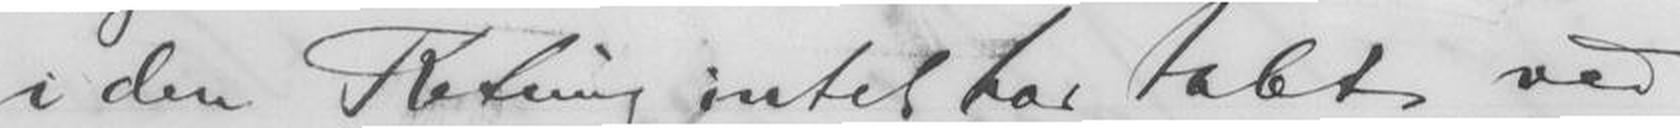i den Retning intet har tabt ved
